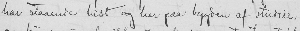har staaende hist og her paa bygden af studier,
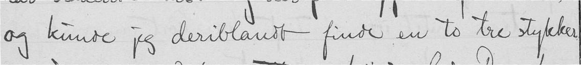og kunde jeg deriblandt finde en to tre stykker
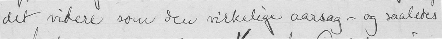det videre som den virkelige aarsag - og saaledes
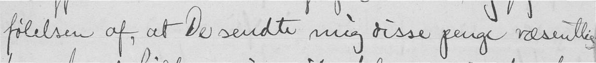følelsen af, at De sendte mig disse penge væsentlig
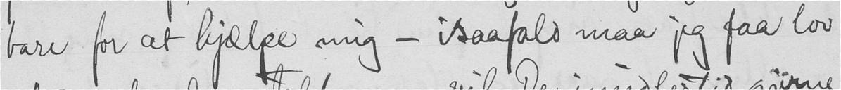bare for at hjælpe mig - isaafald maa jeg faa lov
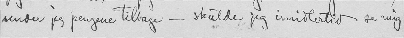sender jeg pengene tilbage - skulde jeg imidlertid se mig
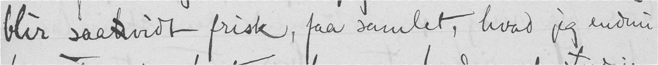blir saavidt frisk, faa samlet, hvad jeg endnu
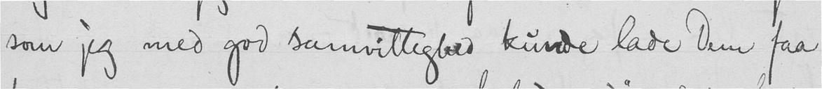som jeg med god samvittighed kunde lade Dem faa
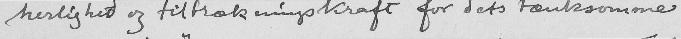herlighed og tiltrækningskraft for dets tænksomme
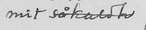mit såkaldte
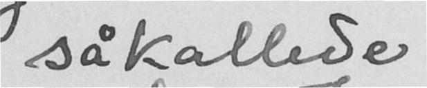såkallede
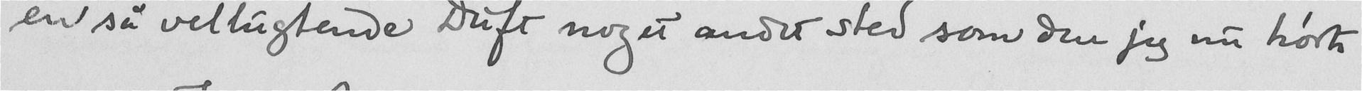en så vellugtende Duft noget andet sted som den jeg nu hørte
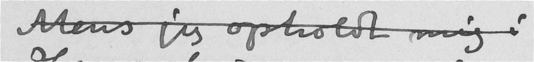Mens jeg opholdt mig i
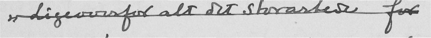"Ligeoverfor alt det storartede for"
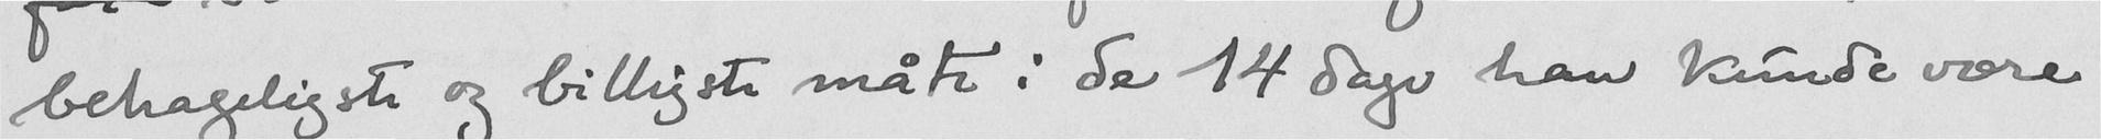behageligste og billigste måte i da 14 dage han kunde være
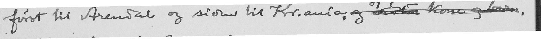først til Arendal og siden til Kr.ania, og mødte kone og barn.
